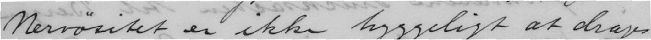Nervøsitet er ikke hyggeligt at drages
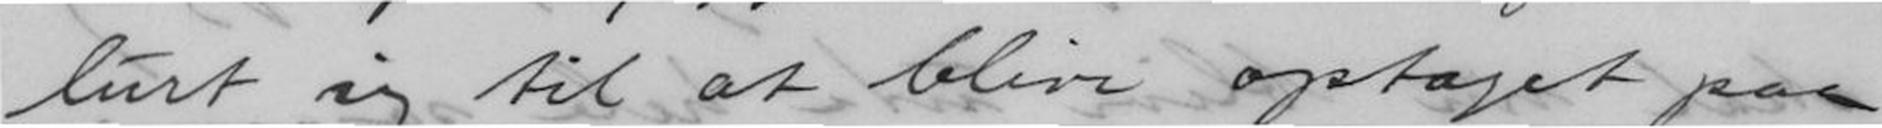lurt sig til at blive optaget paa
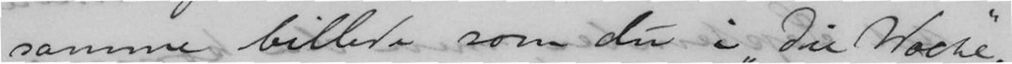samme billede som du i "Die Woche",
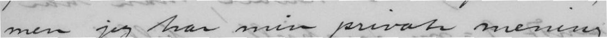men jeg har min private mening
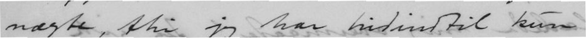nægte, thi jeg har hidindtil kun
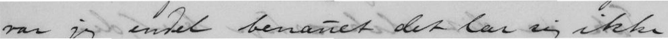var jeg endel benauet det lar sig ikke
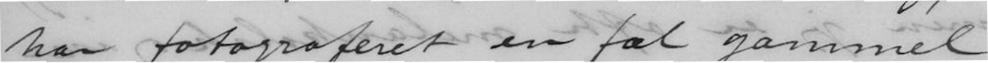har fotograferet en fæl gammel
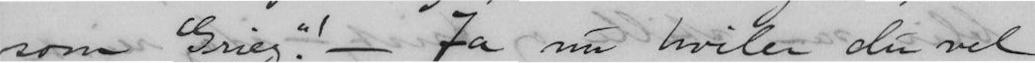som "Grieg"! - Ja nu hviler du vel
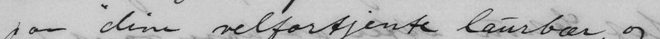paa dine velfortjente laurbær, og
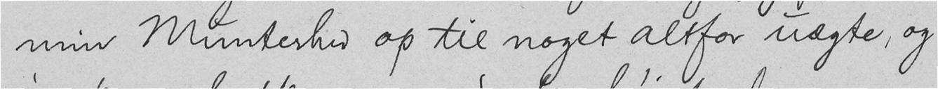min Munterhed op til noget altfor uægte, og
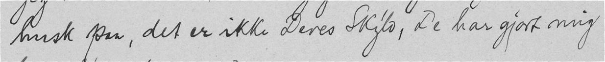husk paa, det er ikke Deres Skyld, De har gjort mig
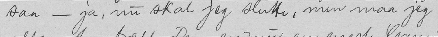saa - ja, nu skal jeg slutte, men maa jeg
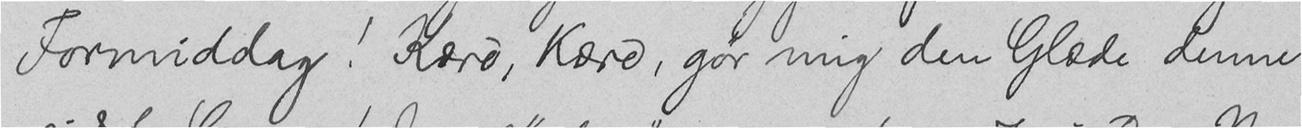Formiddag! Kære, kære, gør mig den Glæde denne
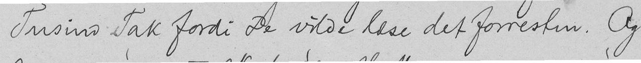Tusind Tak fordi De vilde læse det forresten. Og
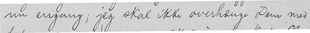nu engang; jeg skal ikke overhænge Dem med
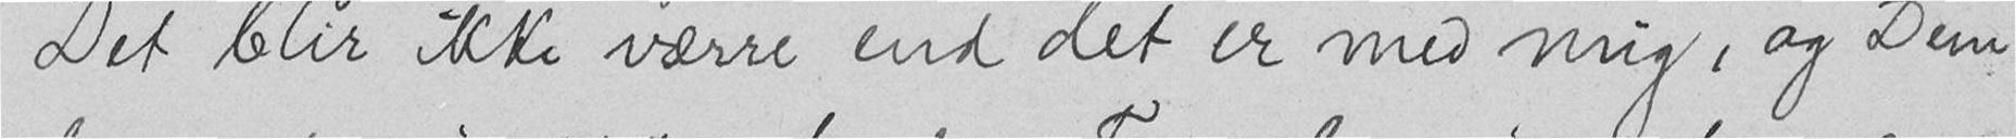Det blir ikke værre end det er med mig, og Dem
